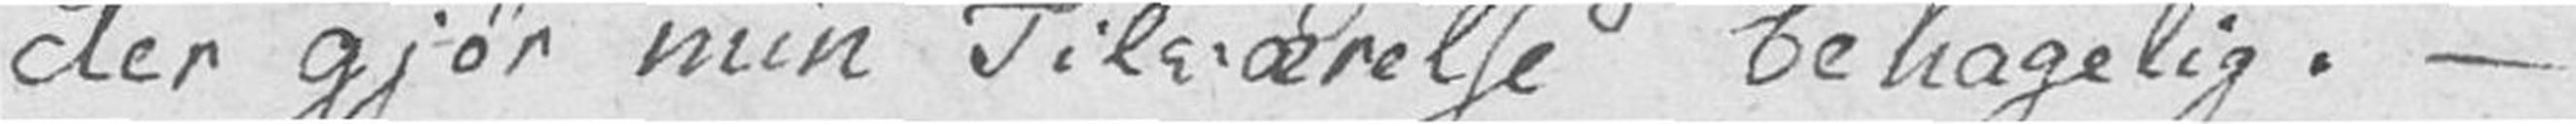der gjør min Tilværelse behagelig. –
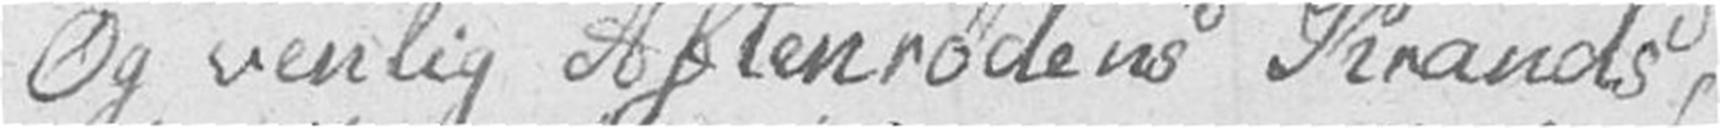Og venlig Aftenrødens Krands,
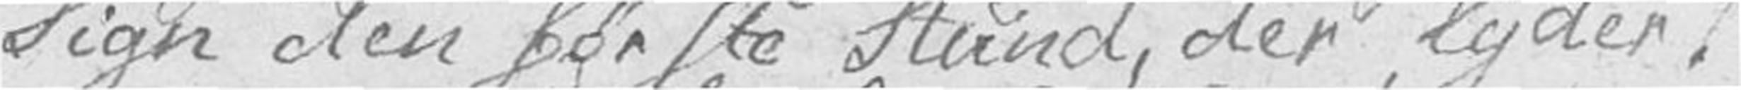Sign den første Stund, der lyder!
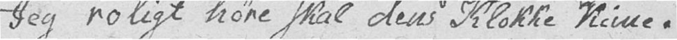Jeg roligt høre skal dens Klokke kime.
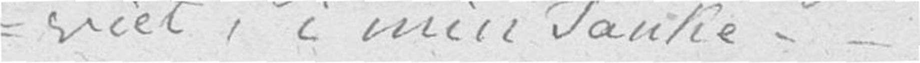-viet, i min Tanke. –
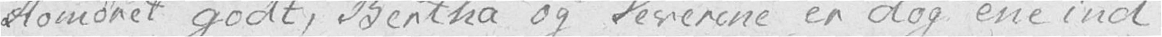Homøret godt, Bertha og Severine er dog ene ind
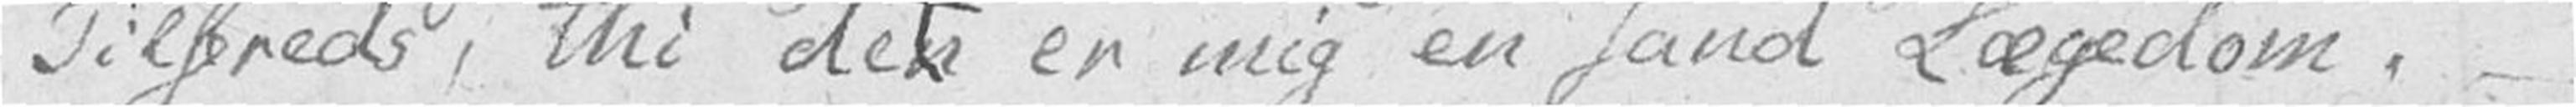Tilfreds, thi det er mig en sand Lægedom. –
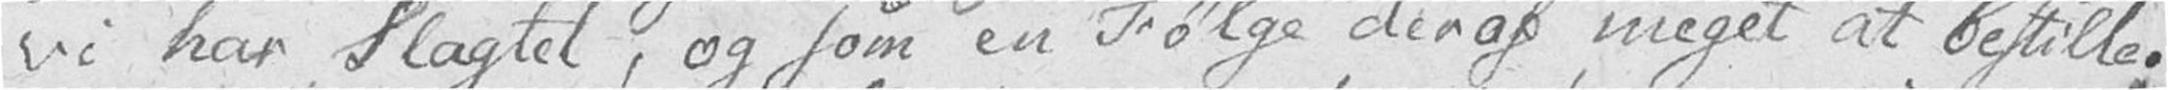vi har Slagtet, og som en Følge deraf meget at bestille.
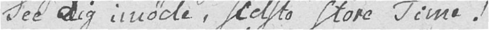See dig imøde, sidste store Time!
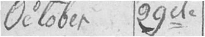October 29de
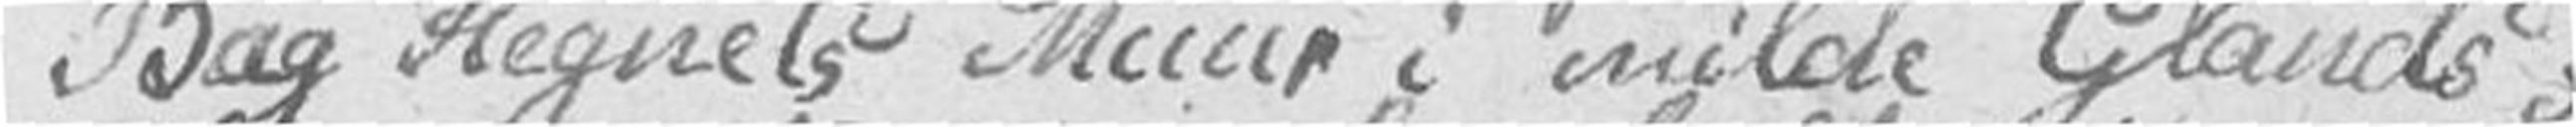Bag Hegnets Muur i milde Glands,
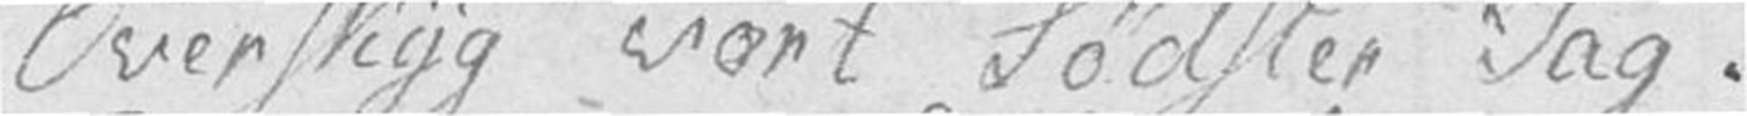Overskyg vort Sødster Tag.
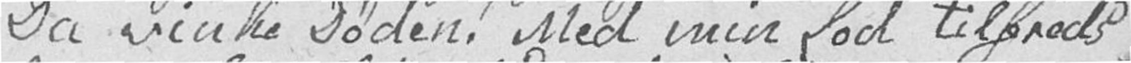Da vinke Døden! Med min Lod tilfreds
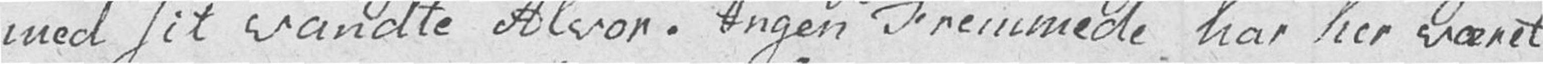med sit vandte Alvor. Ingen Fremmede har her været
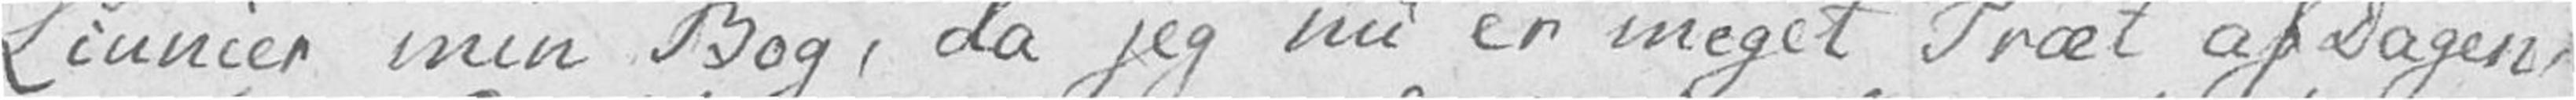Linnier min Bog, da jeg nu er meget Træt af Dagen,
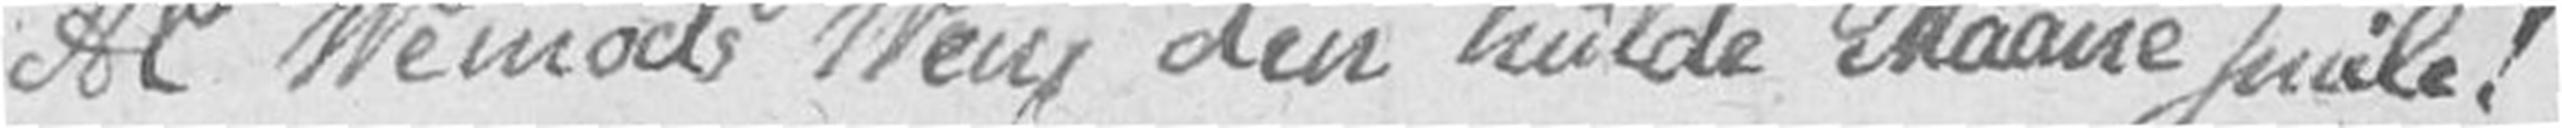Al Vemods Ven, den hulde Maane smile!
